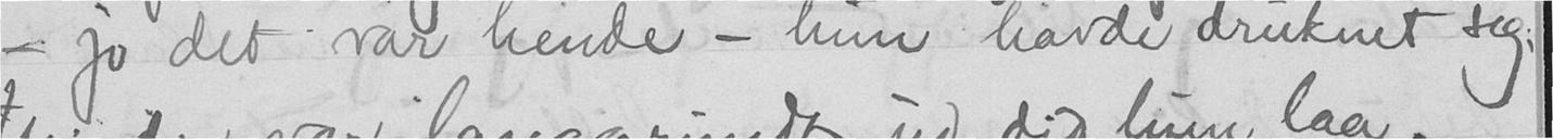- jo det var hende, - hun havde druknet sig;
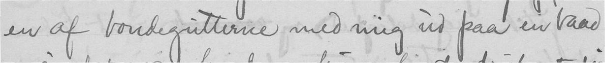en af bondegutterne med mig ud paa en baad
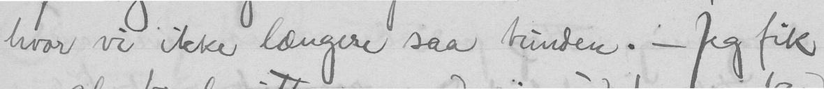hvor vi ikke længere saa bunden. - Jeg fik
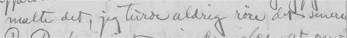malte det, jeg turde aldrig røre det senere.
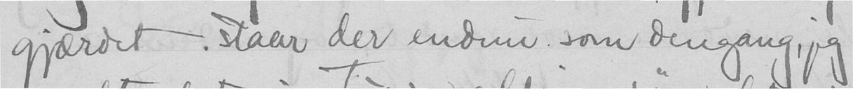gjærdet staar der endnu, som dengang, jeg
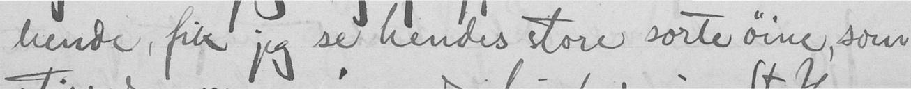hende, fik jeg se hendes store sorte øine, som
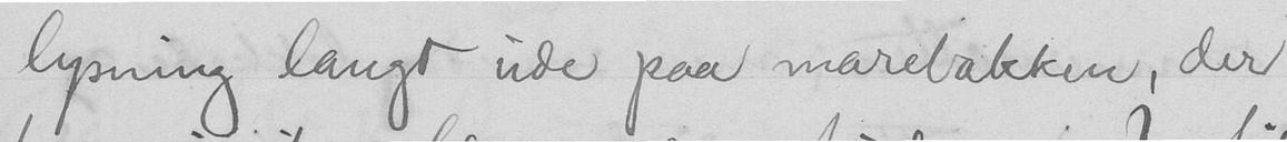lysning langt ude paa marebakken, der
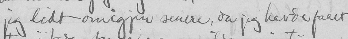jeg lidt omigjen senere, da jeg havde faaet
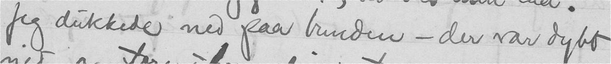Jeg dukkede ned paa bunden - der var dybt
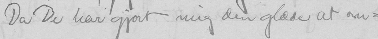Da De har gjort mig den glæde at om-
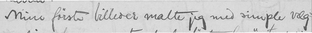Mine første billeder malte jeg med simple væg-
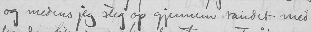og medens jeg steg op gjennem vandet med
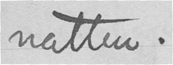natten.
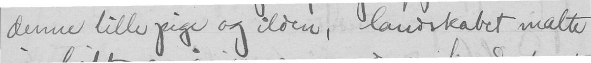denne lille pige og ilden, - landskabet malte
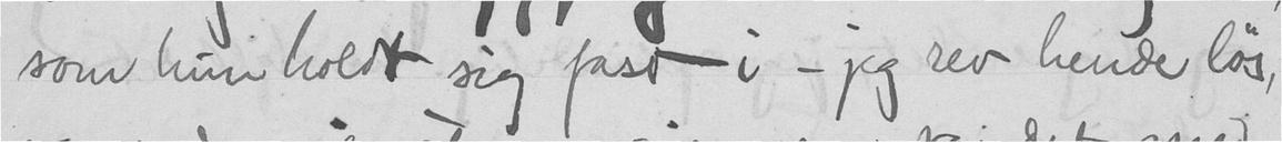som hun holdt sig fast i - jeg rev hende løs,
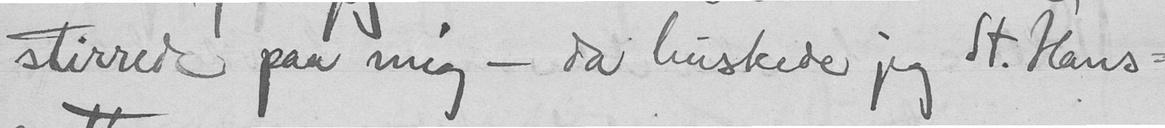stirrede paa mig - da huskede jeg St. Hans-
